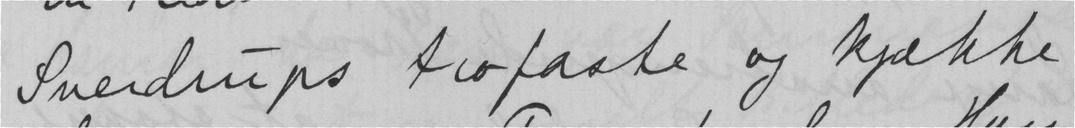Sverdrups trofaste og kjække
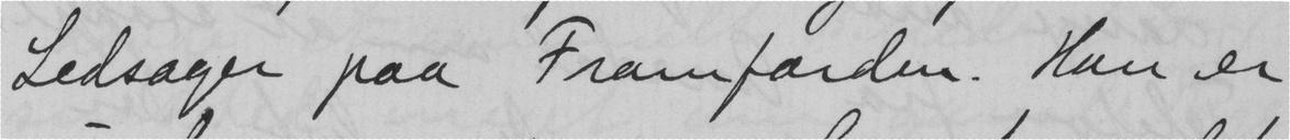Ledsager paa Framfærden. Han er
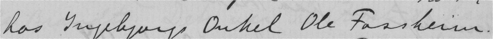hos Ingebjorgs Onkel Ole Fossheim.
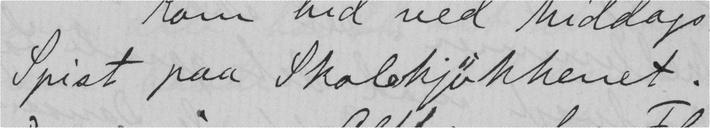Spist paa Skolekjøkkenet.
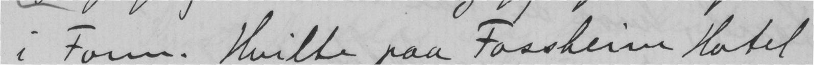i Form. Hvilte paa Fossheim Hotel
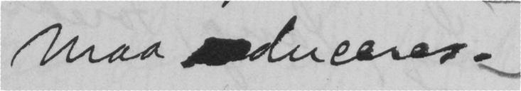maa duceres.
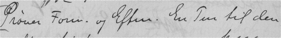Prøven Form. og Eften. En Tur til den
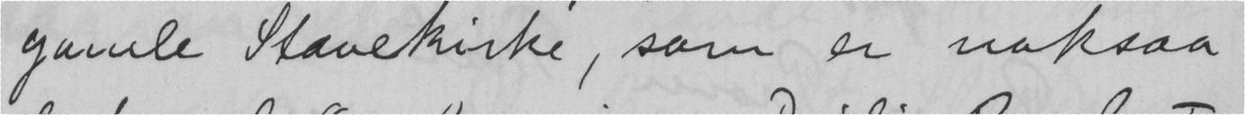gamle Stavekirke, som er noksaa
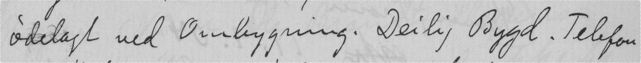ødelagt ved Ombygning. Deilig Bygd. Telefon
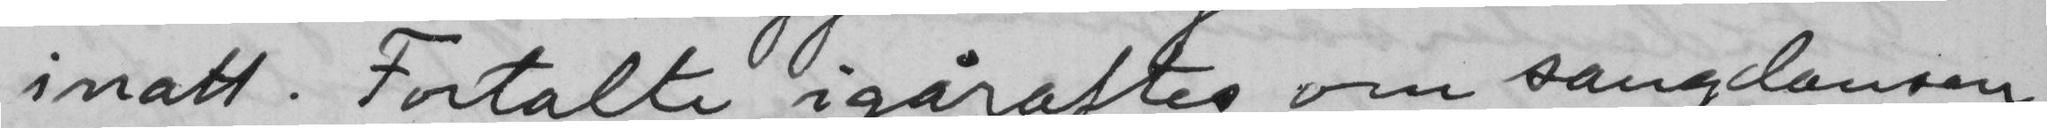inatt. Fortalte igåraftes om sangdansen
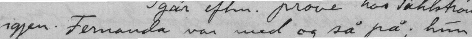igjen. Fernanda var med og så på; hun
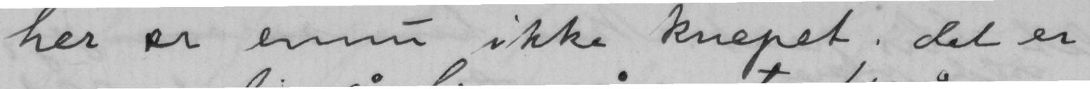her er ennu ikke knepet; det er
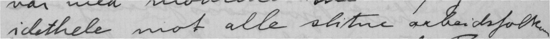idethele mot alle slitne arbeidsfolkene
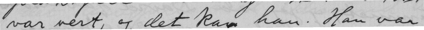var vert, og det kan han. Han var
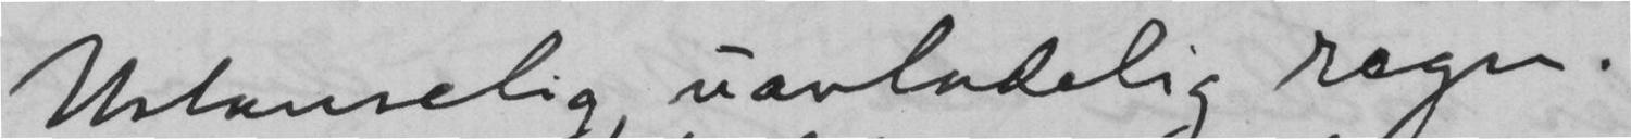Ustanselig, uavladelig regn.
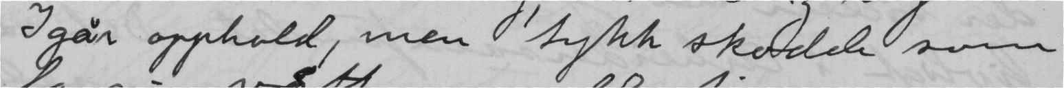Igår opphold, men tykk skodde som
